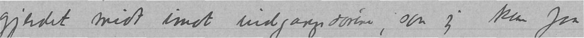gjerdet midt imot indgangsdørene, saa jeg kan faa
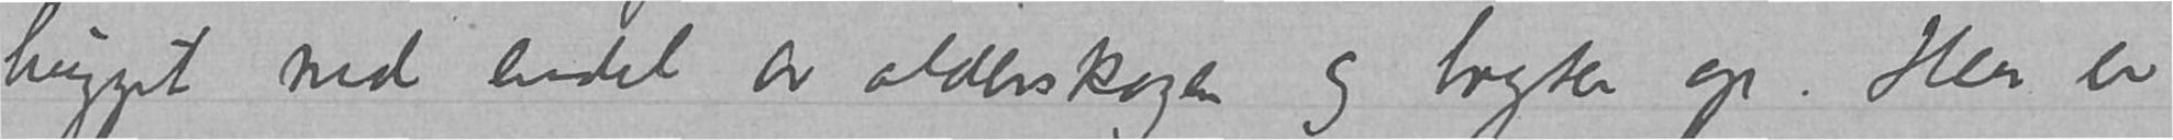hugget ned endel av olderskogen og bryter op.Her er
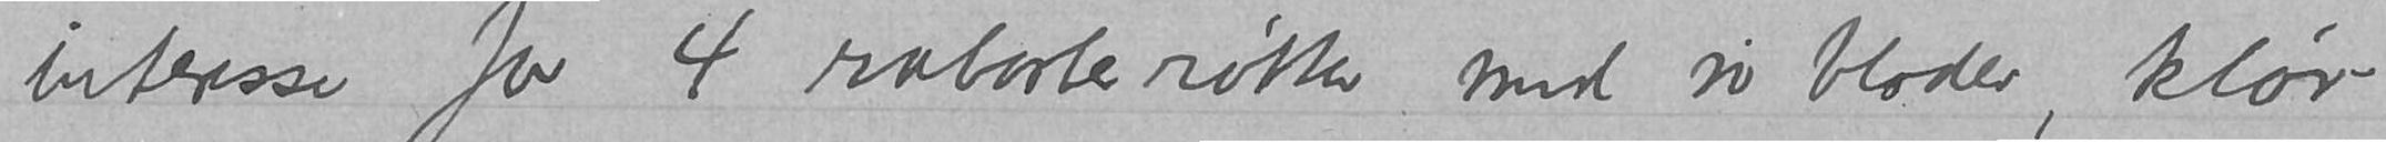interesse for 4 rabarbrarøtter med ni blader, klør
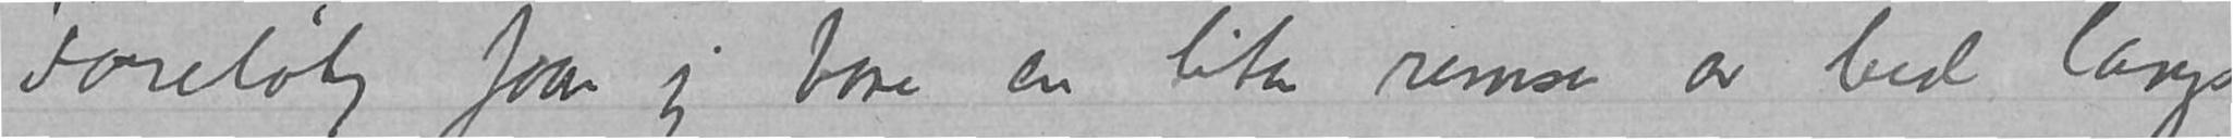Foreløbig faar jeg bare en liten remse av bed langs
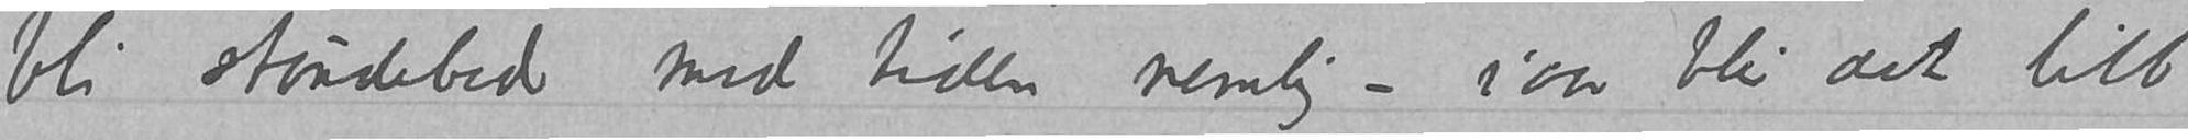bli staudebed med tiden nemlig – iaar blir det litt
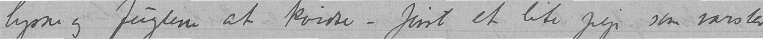lysne og fuglene at kvidre – først et lite pip som varsler
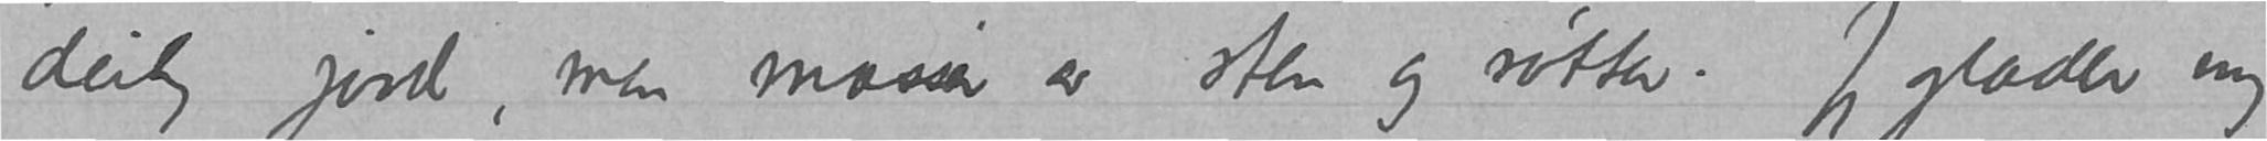deilig jord, men masser av sten og røtter.  Jeg glæder mig
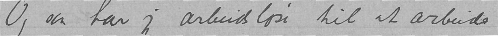Og saa har jeg arbeidsløse til at arbeide.
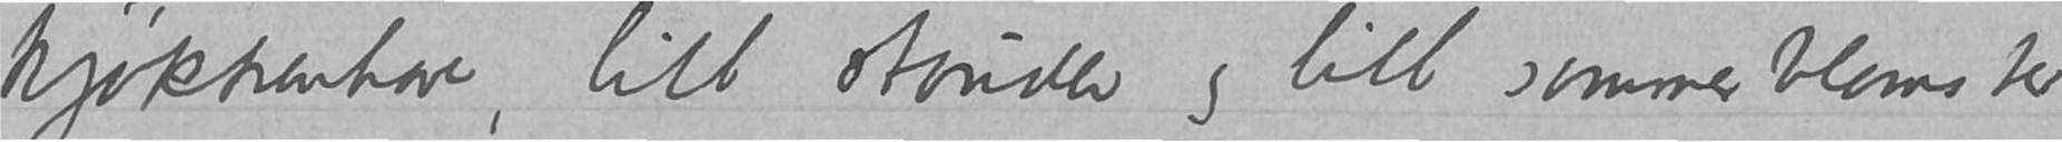kjøkkenhave,  litt stauder og littll sommerblomster
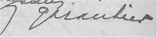og garantier
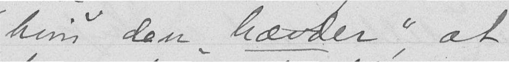hvori den "hævder", at
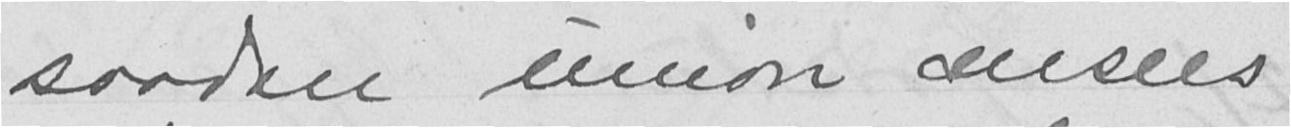saadan union ansees
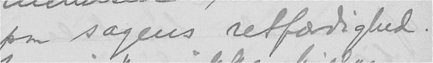paa sagens retfærdighed.
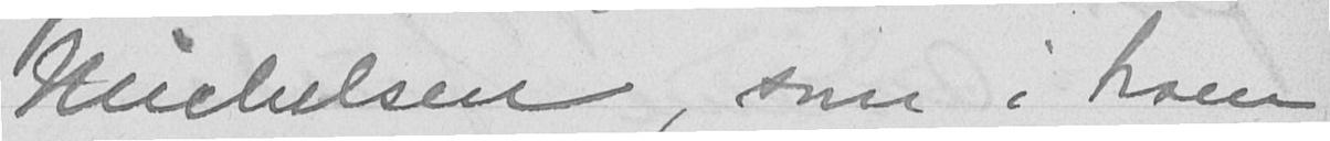Michelsen, som i troen
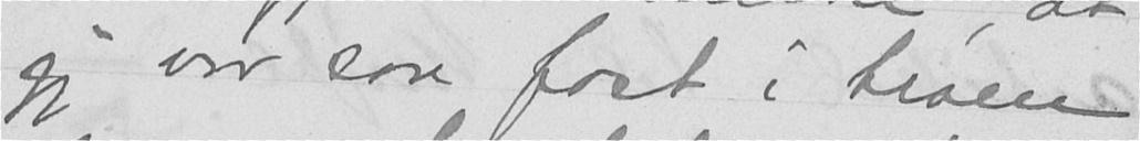jeg var saa fast i troen
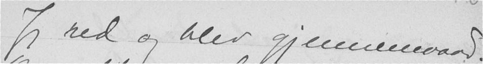Jeg red og blev gjennemvaad.
